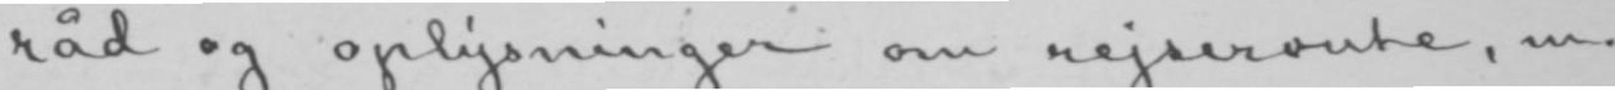råd og oplysninger om rejseroute, m.
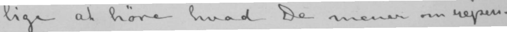lige at høre hvad De mener om rejsen.
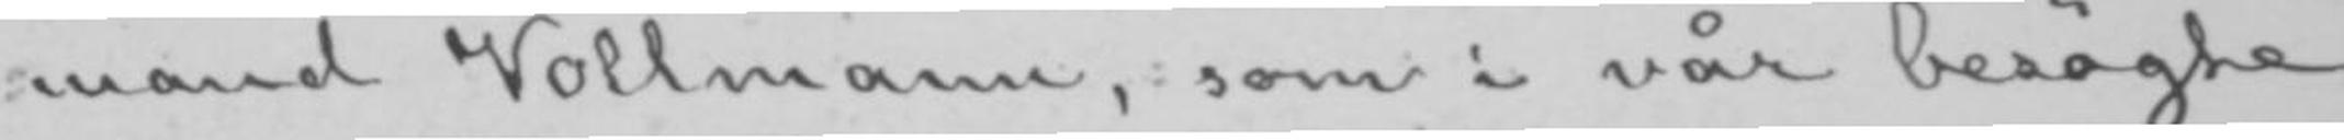mand Vollmann, som i vår besøgte
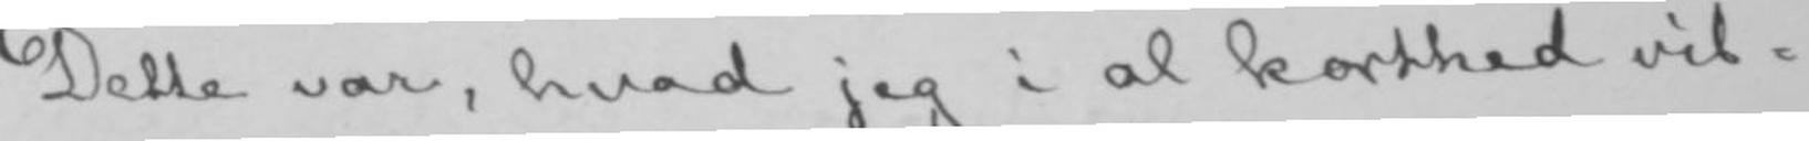Dette var, hvad jeg i al korthed vil-
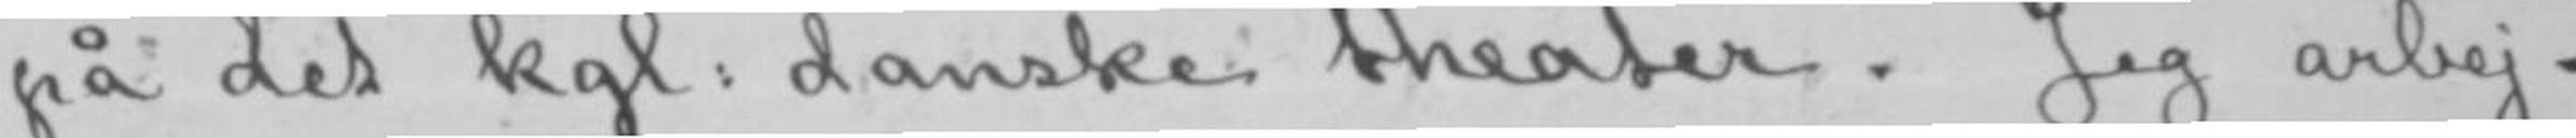på det kgl: danske theater. Jeg arbej-
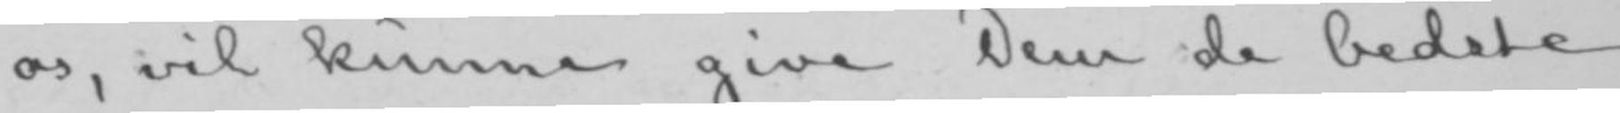os, vil kunne give Dem de bedste
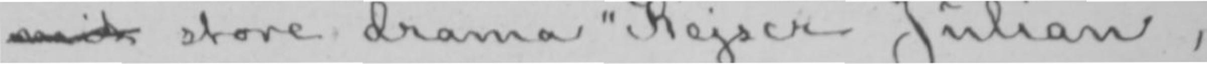mit store drama «Kejser Julian»,
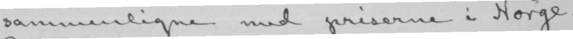sammenligne med priserne i Norge.
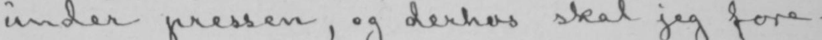under pressen, og derhos skal jeg fore-
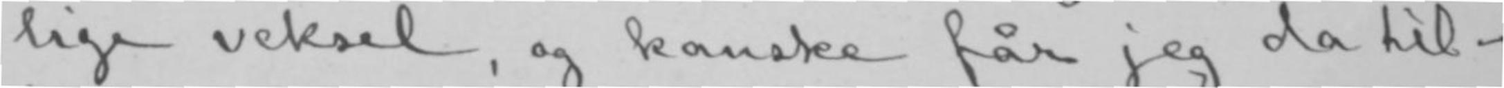lige veksel, og kanske får jeg da til-
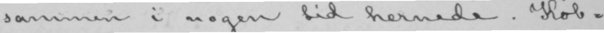sammen i nogen tid hernede. Køb-
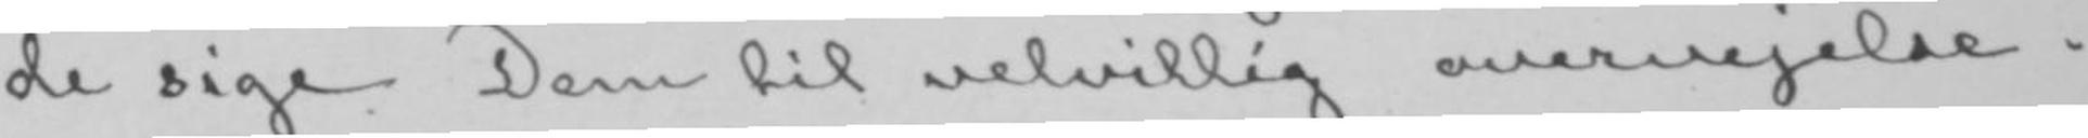de sige Dem til velvillig overvejelse.
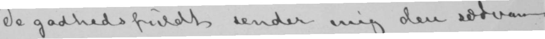De godhedsfuldt sender mig den sædvan-
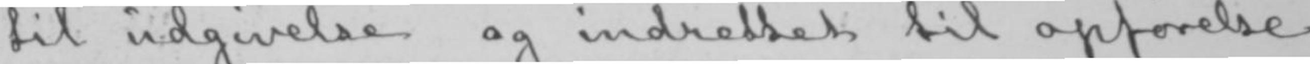til udgivelse og indrettet til opførelse
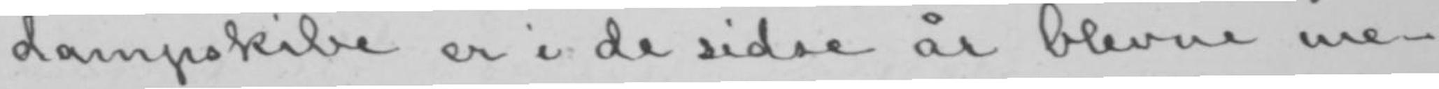dampskibe er i de sidse år blevne me-
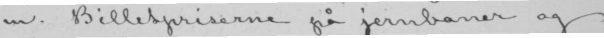m. Billetpriserne på jernbaner og
